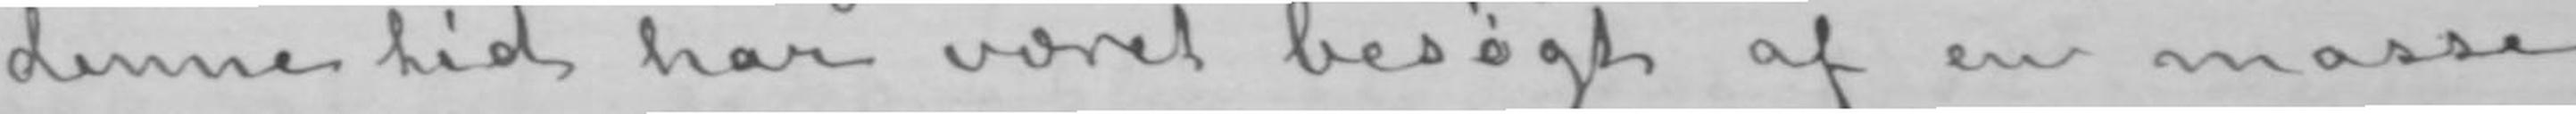denne tid har været besøgt af en masse
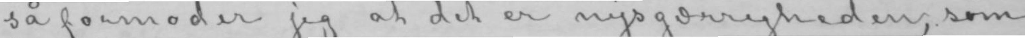så formoder jeg at det er nysgærrigheden, som
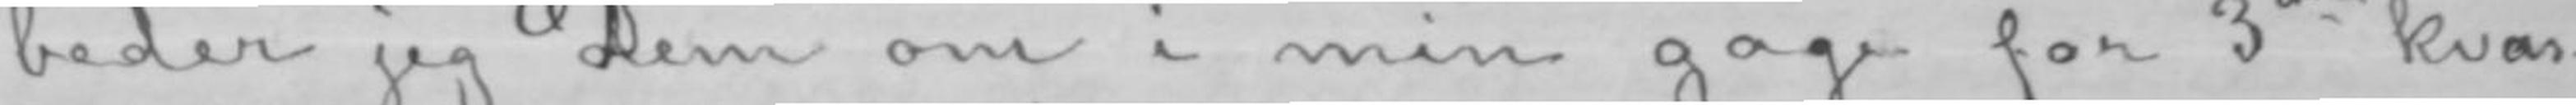beder jeg Dem om i min gage for 3die kvar-
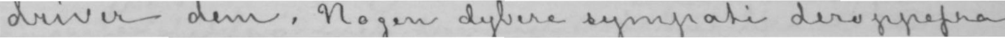driver dem. Nogen dybere sympati deroppefra
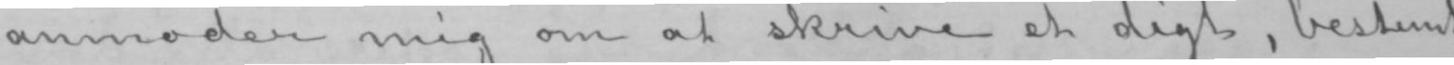anmoder mig om at skrive et digt, bestemt
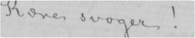Kære svoger!
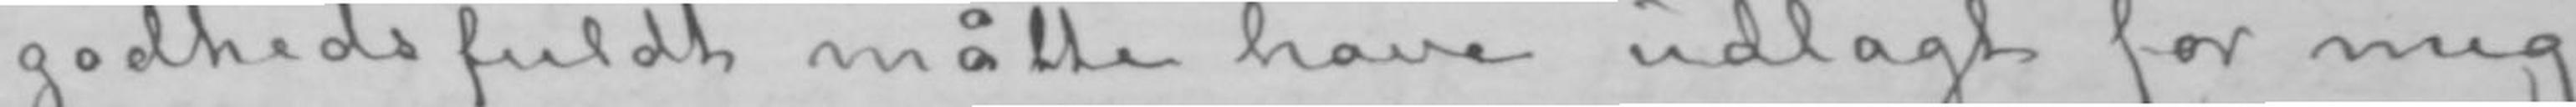godhedsfuldt måtte have udlagt for mig
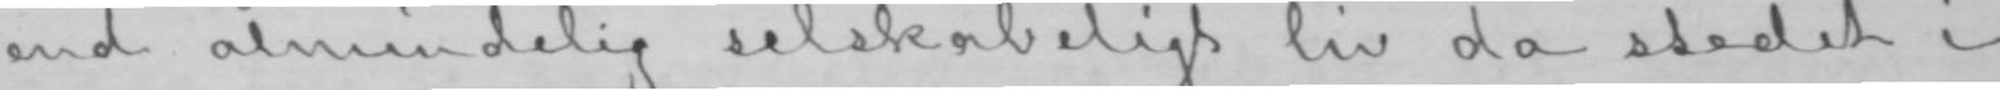end almindelig selskabeligt liv da stedet i
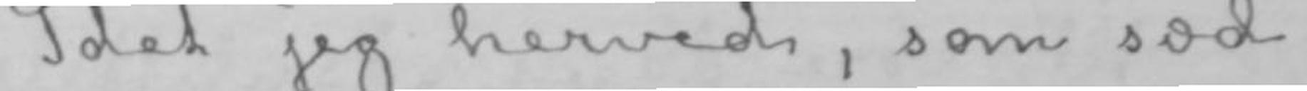Idet jeg herved, som sæd-
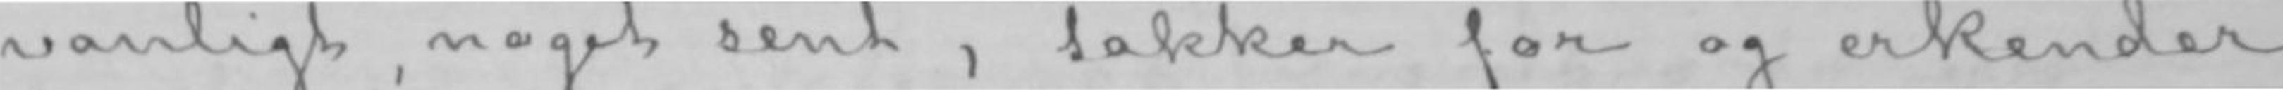vanligt, noget sent, takker for og erkender
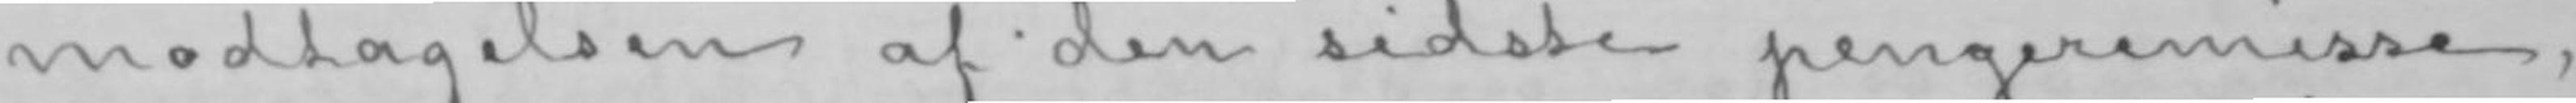modtagelsen af den sidste pengeremisse,
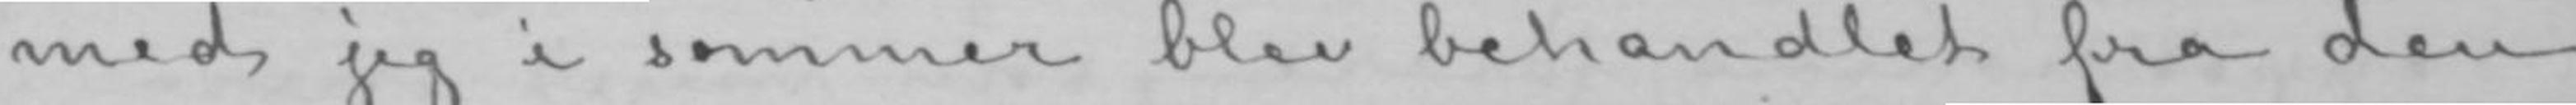med jeg i sommer blev behandlet fra den
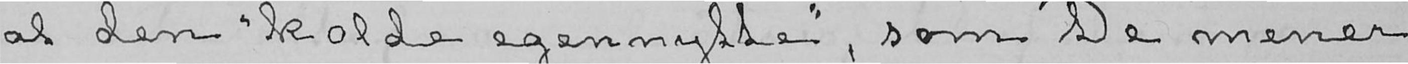at den «kolde egennytte», som De mener
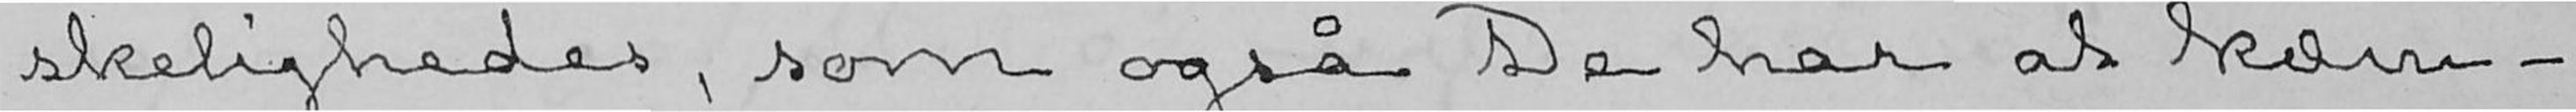skeligheder, som også De har at kæm-
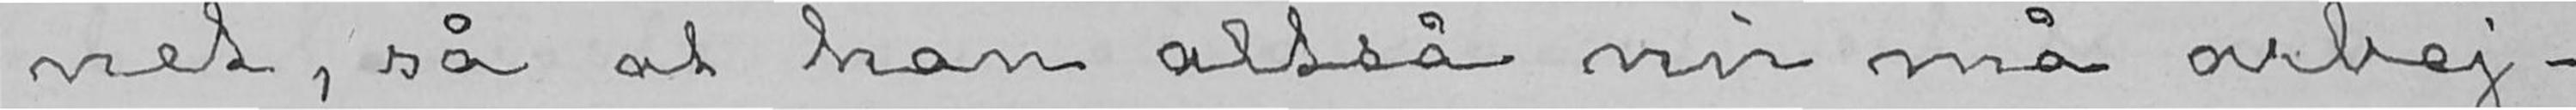net, så at han altså nu må arbej-
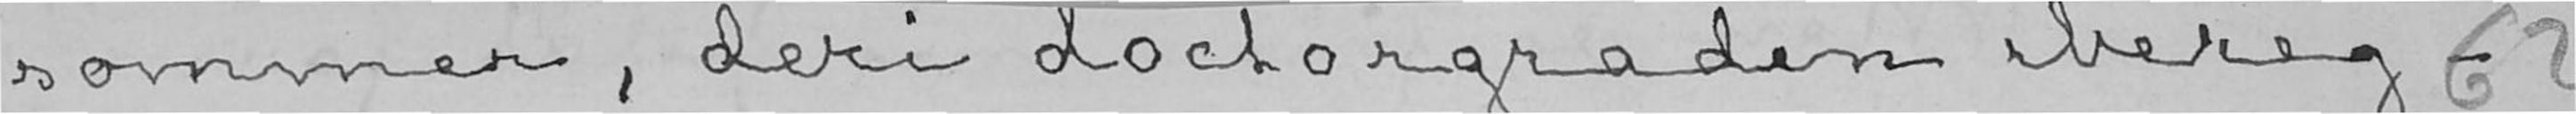sommer, deri doctorgraden ibereg-
